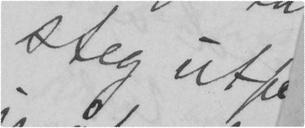steg utfe
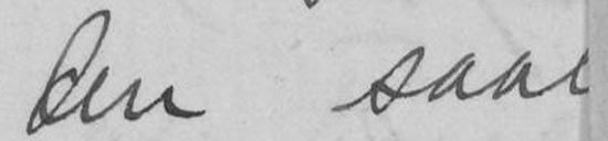an saar
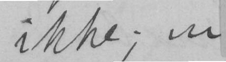ikke; ei
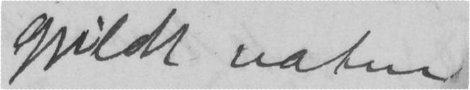gjildt natur
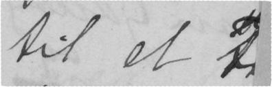til et Fr
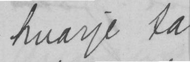hvarje ta
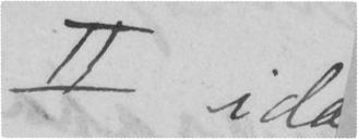I ida
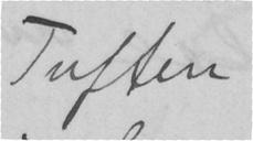Tuffen
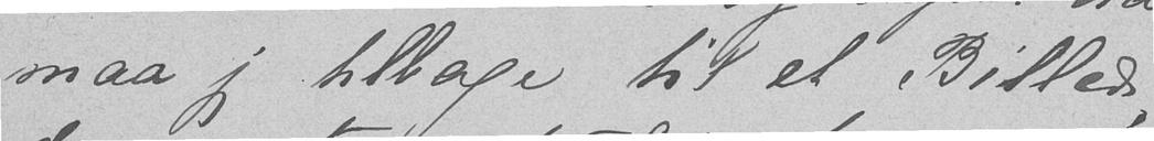maa jeg tilbage til et Billede,
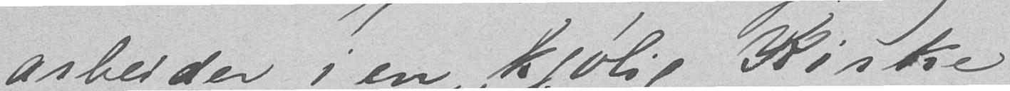arbeider i en kjølig Kirke
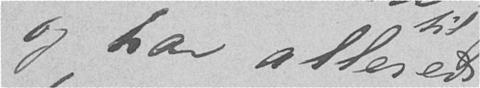og har allerede
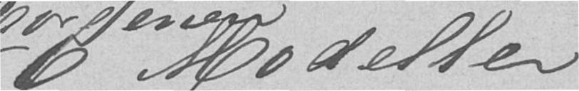6 Modeller
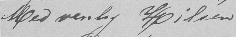Med venlig Hilsen
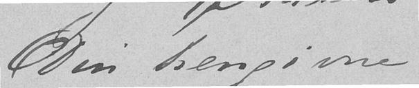Din hengivne
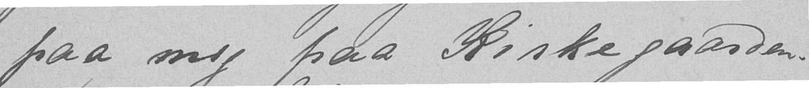paa mig paa Kirkegaarden.
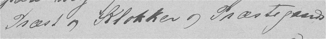Præst og Klokker og Præstegaards
